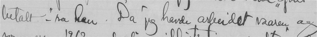betalt" - sa han. Da jeg havde arbeidet vaaren og
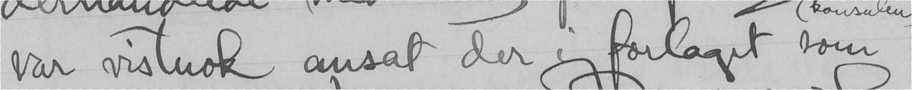var vistnok ansat der, i forlaget som
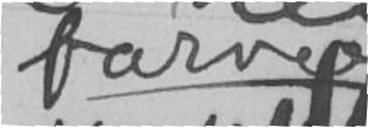farver
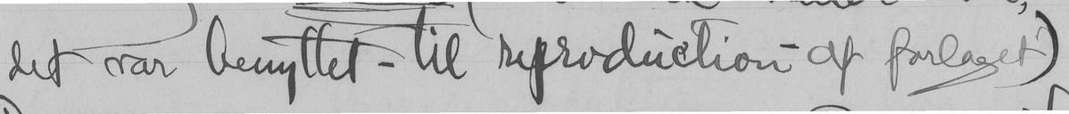det var benyttet - til reproduction - at forlaget)
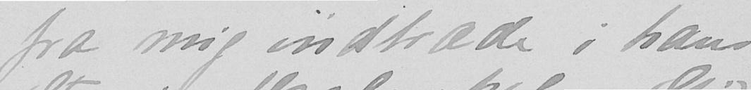fra mig indtræde i hans
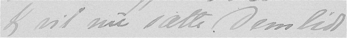Jeg vil nu sætte Dem lidt
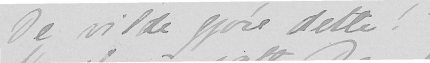De vilde gjøre dette!
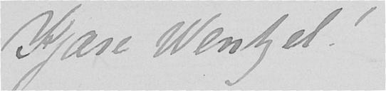Kjære Wentzel!
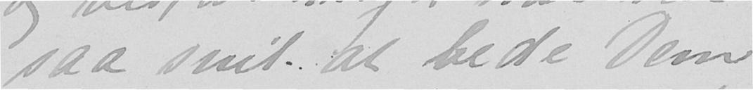saa snil at bede Dem
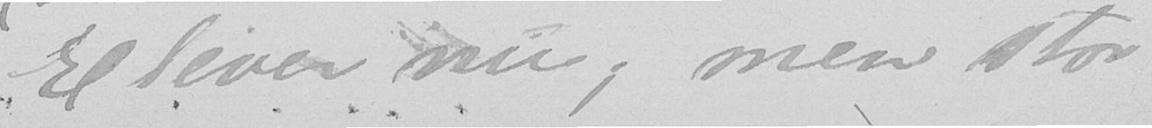Elever nu; men stor
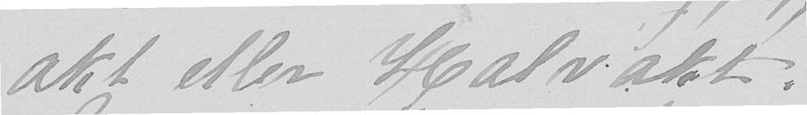akt eller Halvakt.
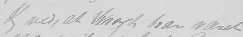Jeg ved, at Krogh har været
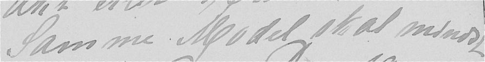Samme Model skal mindst
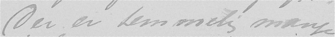Der er temmelig mange
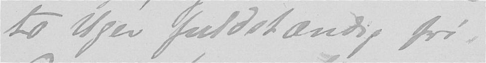to Uger fuldstændig fri.
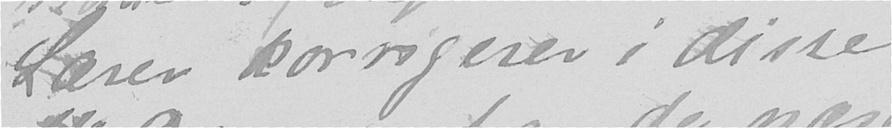Lærer korrigerer i disse
